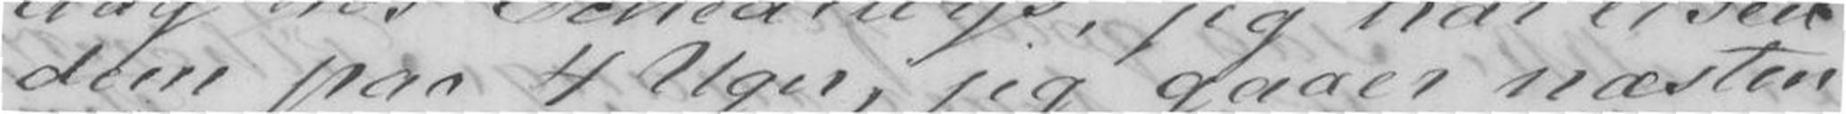dem paa 4 Uger, jeg gaaer næsten
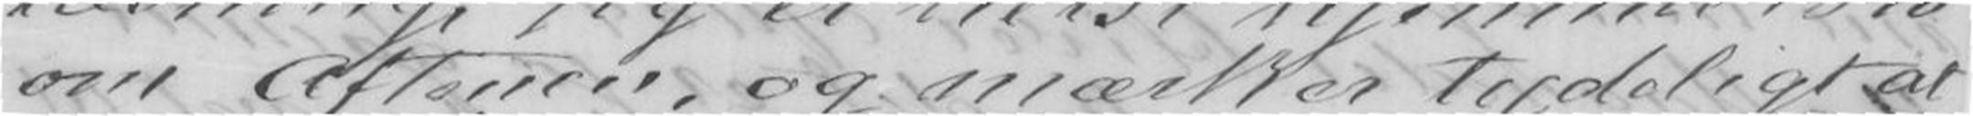om Aftenen, og mærker tydelig at
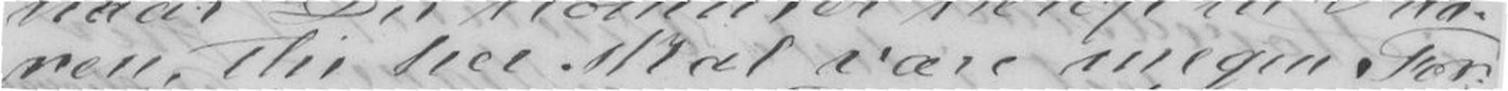ren, thi her skal være megen For-
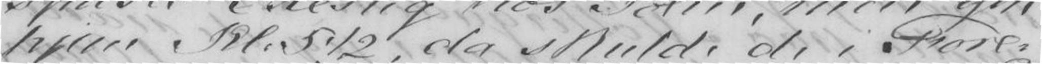hjem Kl 5½, da skulde de i Fore-
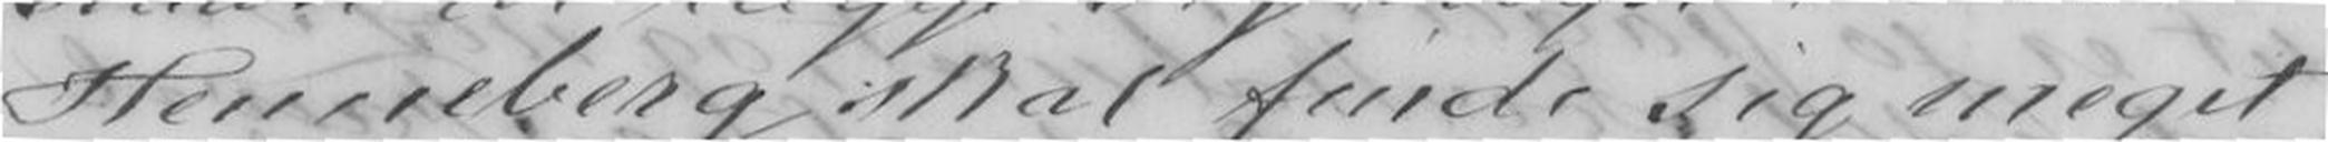Henneberg skal finde sig meget
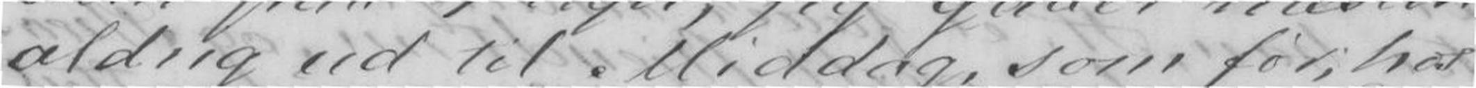aldrig ud til Middag, som før, hos
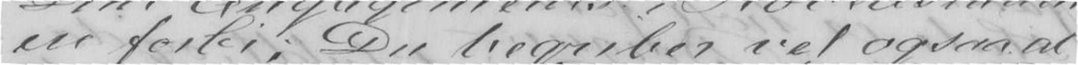ere forbi; Du begriber vel ogsaa at
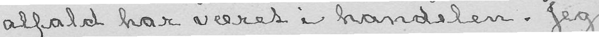alfald har været i handelen. Jeg
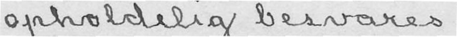opholdelig besvares.
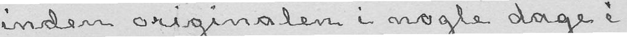inden originalen i nogle dage i-
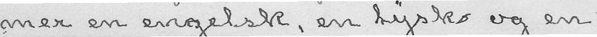mer en engelsk, en tysk og en
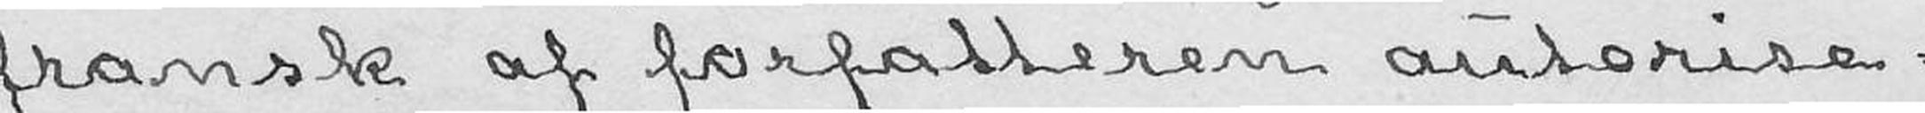fransk af forfatteren autorise-
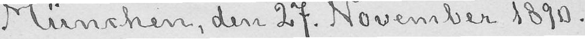München, den 27. November 1890.
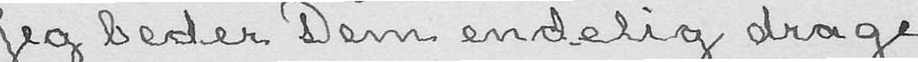Jeg beder Dem endelig drage
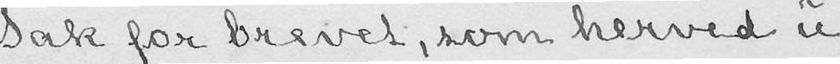Tak for brevet, som herved u-
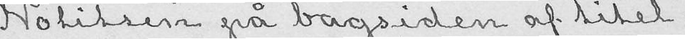Notitsen på bagsiden af titel-
